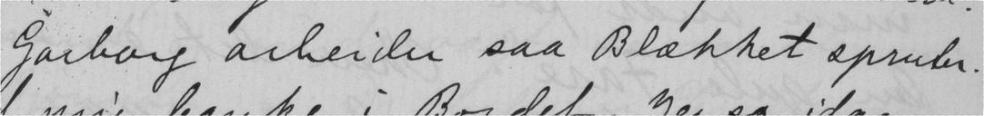Garborg arbeider saa Blækket spruter.
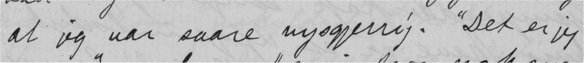at jeg var saare nysgjerrig. "Det er jeg
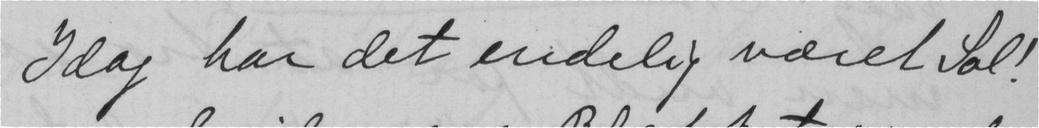Idag har det endelig været Sol!
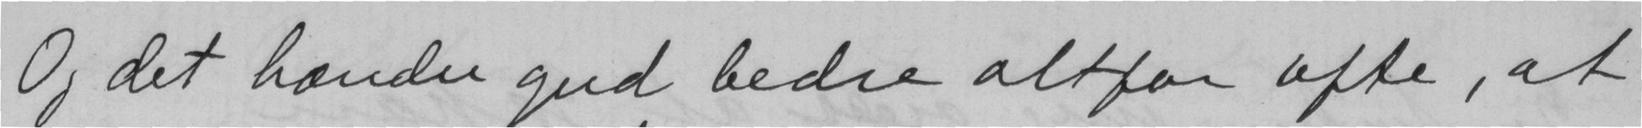Og det hænder gud bedre altfor ofte, at
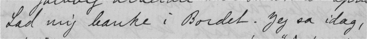Lad mig banke i Bordet. Jeg sa idag,
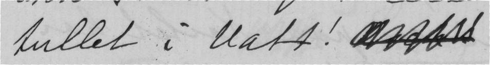tullet i vatt!
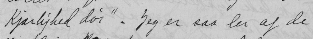Kjærlighed dør". Jeg er saa lei af de
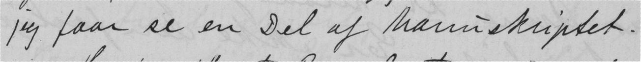jeg faar se en Del af Manuskriptet.
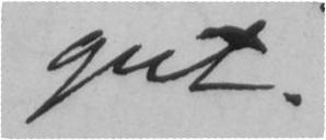gut.
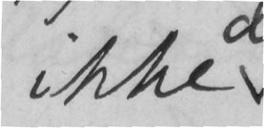ikke
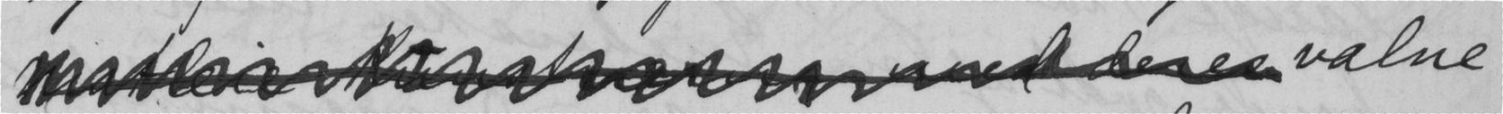valne
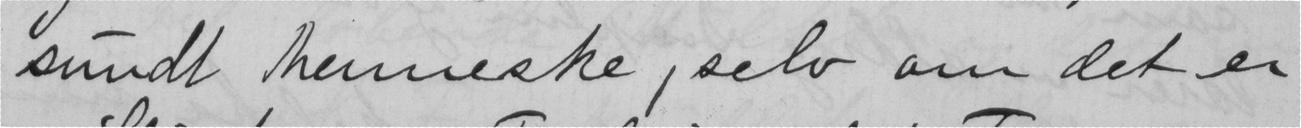sundt menneske, selv om det er
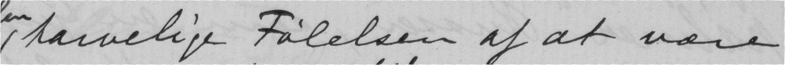tarvelige Følelsen af at være
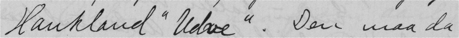Haukland "Udve". Den maa da
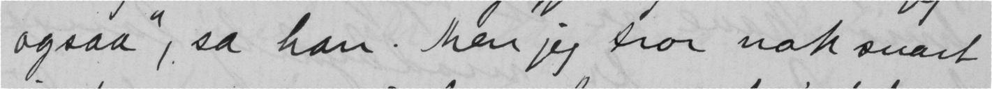ogsaa", sa han. Men jeg tror nok snart
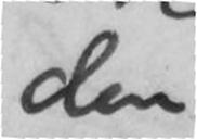den
